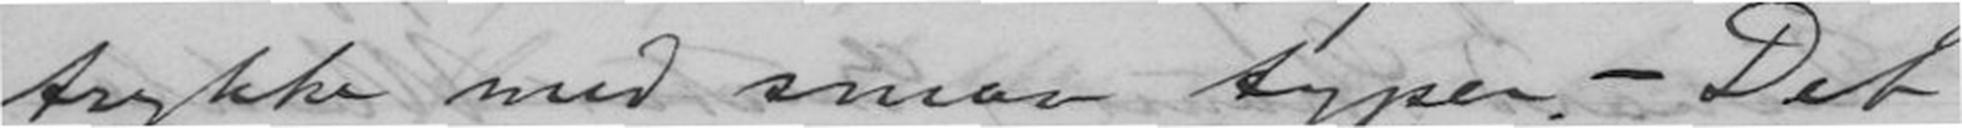trykke med smaa typer. - Det
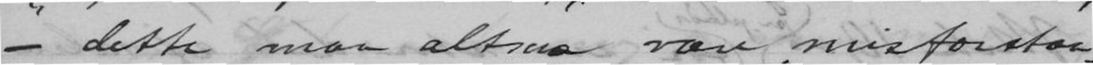- dette maa altsaa være "misforstaa-
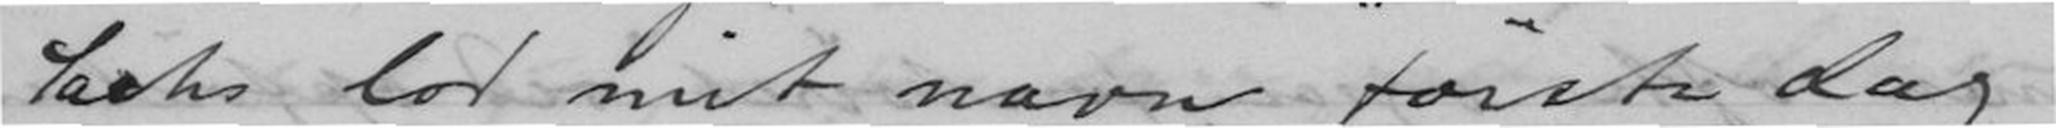Sachs lod mit navn første dag
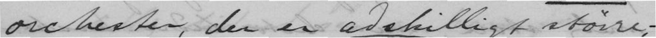orchester, der er adskilligt større, -
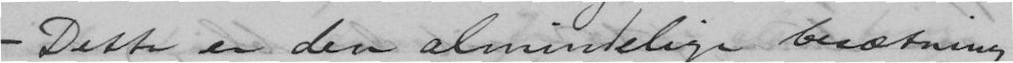- Dette er den almindelige besætning
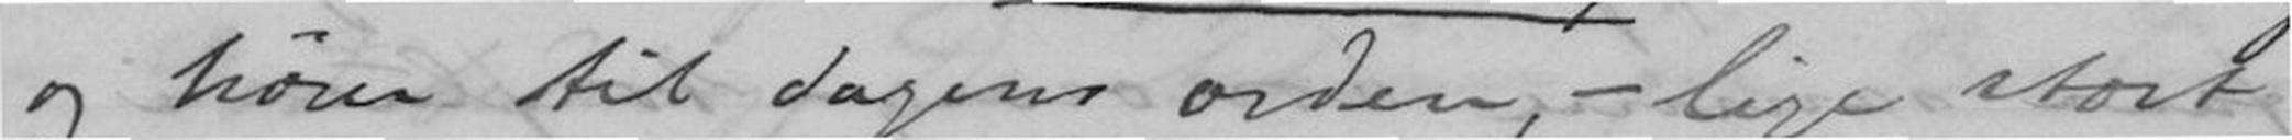og hører til dagens orden, - lige stort
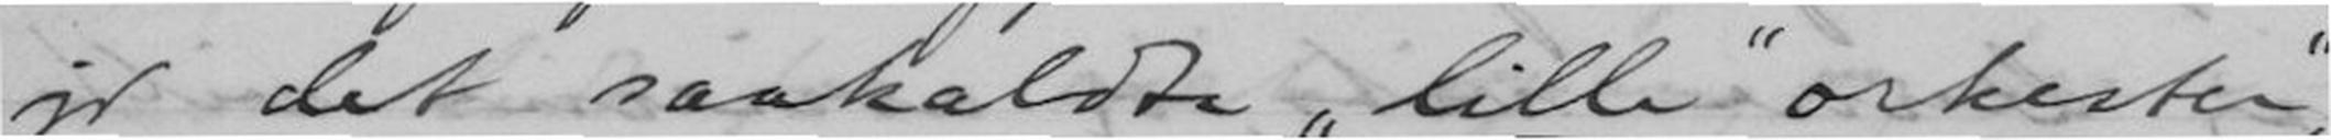jo det saakaldte "lille" orkester",
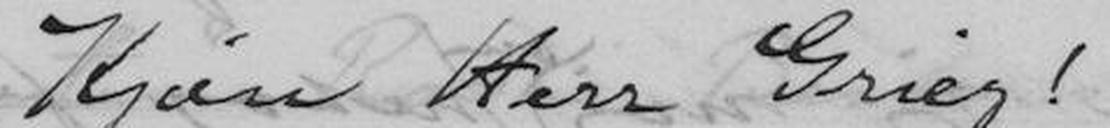Kjære Her Grieg!
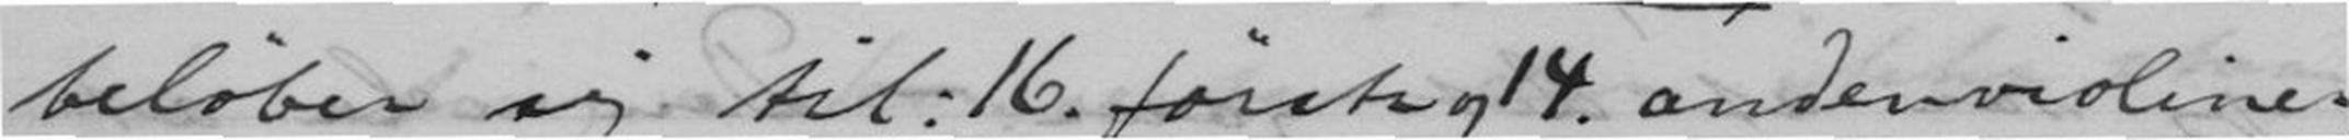beløber sig til: 16. første og 14. andenvioliner,
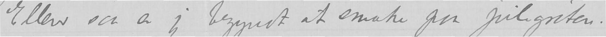Ellers saa er jeg begyndt at smake paa julegrøten.
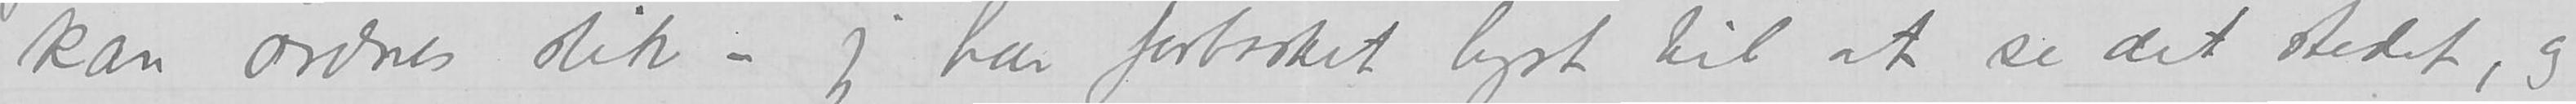kan ordnes slik – jeg har forbasket lyst til at se det stedet, og
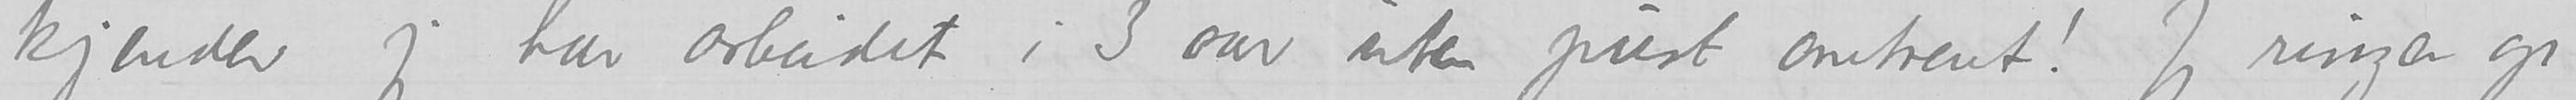kjender jeg har arbeidet i 3 aar uten pust omtrent! Jeg ringer op
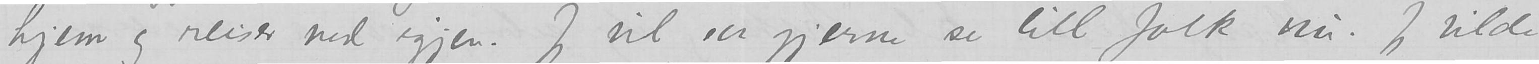hjem og reiser ned igjen. Jeg vil saa gjerne se litt folk nu. Jeg vilde
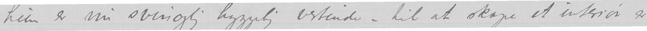hun er nu svinagtig hyggelig vertinde – til at skape et interiør er
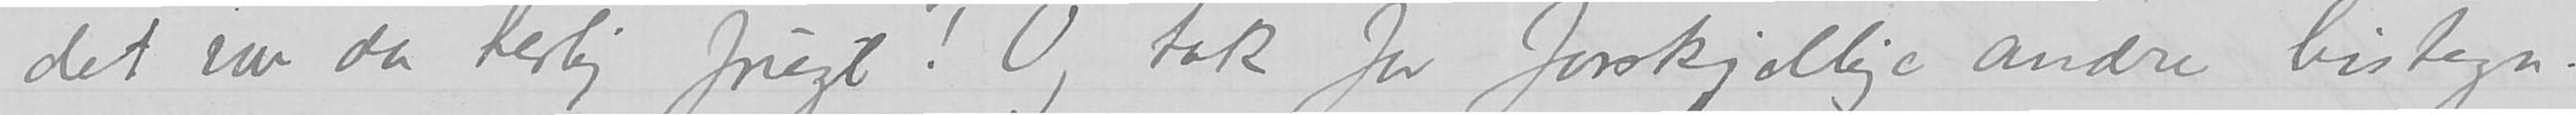det var da herlig frugt! Og tak for forskjellige andre livstegn.
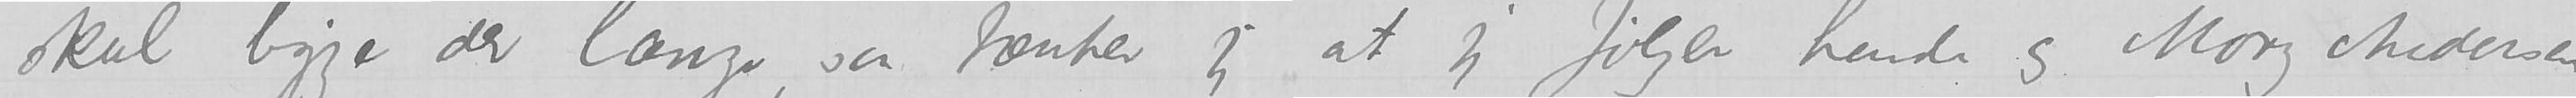skal ligge der længe, saa tænker jeg at jeg følger hende og Mary Andersen
